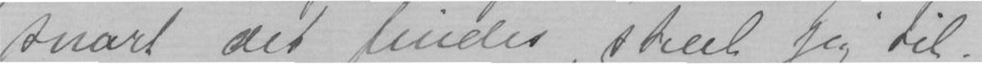snart det findes skal jeg til-
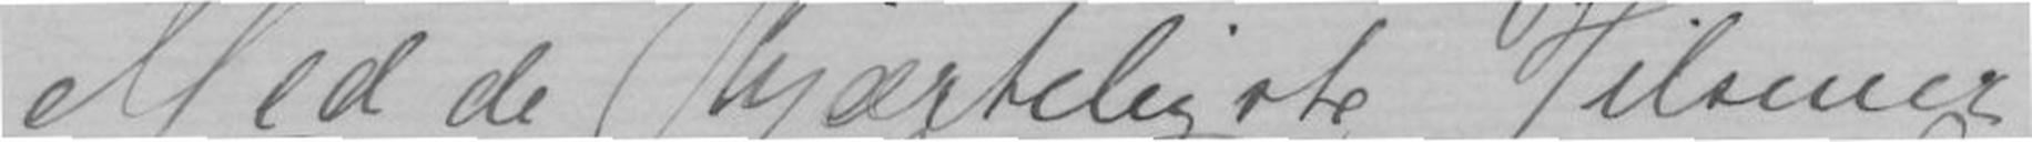Med de kjærligste Hilsener
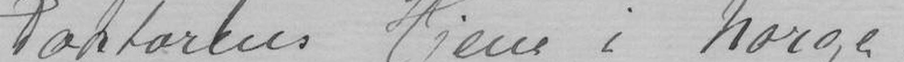Doktorens Hjem i Norge.
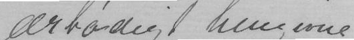ærbødigst hengivne
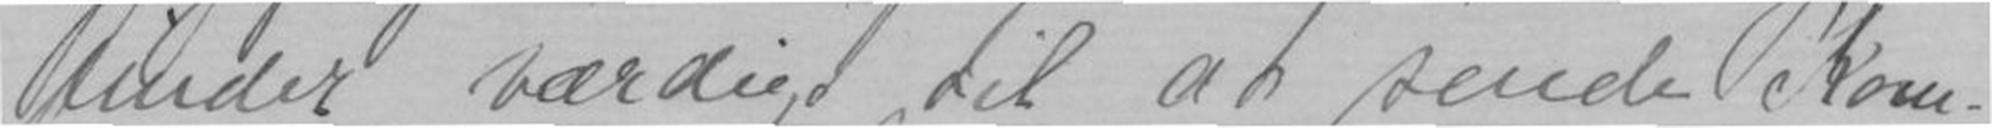finder værdigt til at sende Kom-
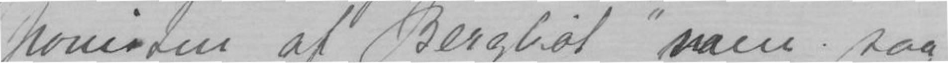ponisten af Bergliot, men saa
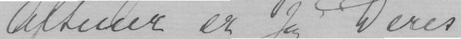Aftener er jeg Deres
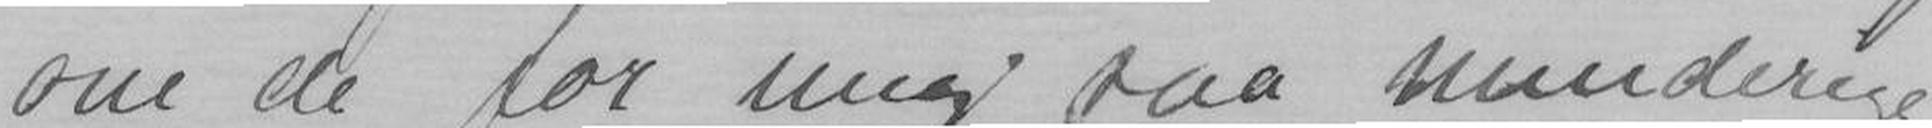om de for mig saa minderige
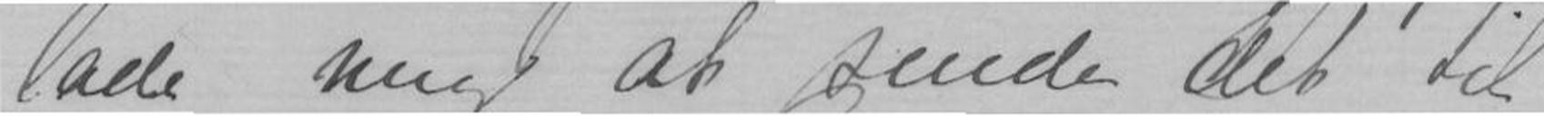lade mig at sende det til
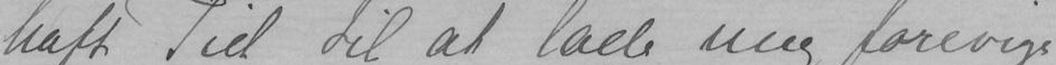haft Tid til at lade mig forevige,
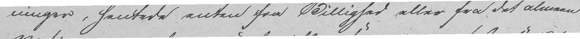ninger, hentede enten fra Billighed eller fra det almeen
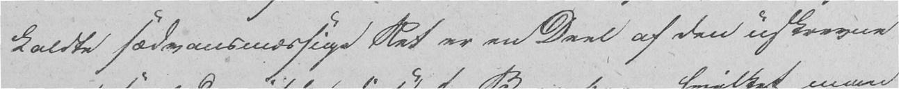kaldte sædvansmæssige Ret er en Deel af den uskrevne
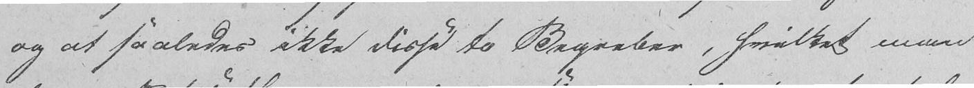og at saaledes ikke disse to Begreber, hvilket man
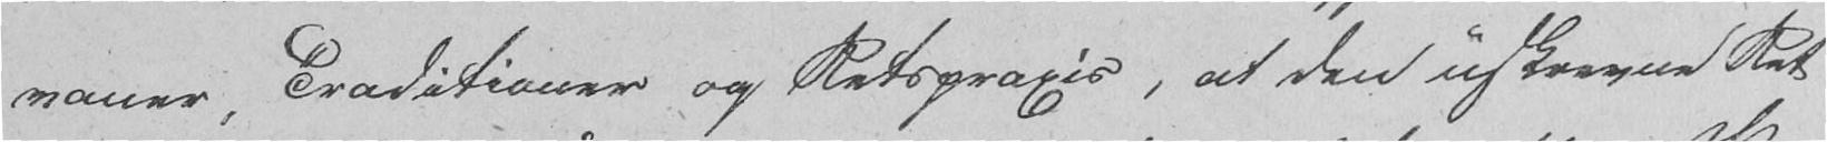vaner, Traditioner og Retspraxis, at den uskrevne Ret
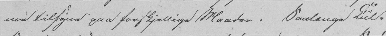me tilsyne paa forskjellige Maader. Saalænge Kul-
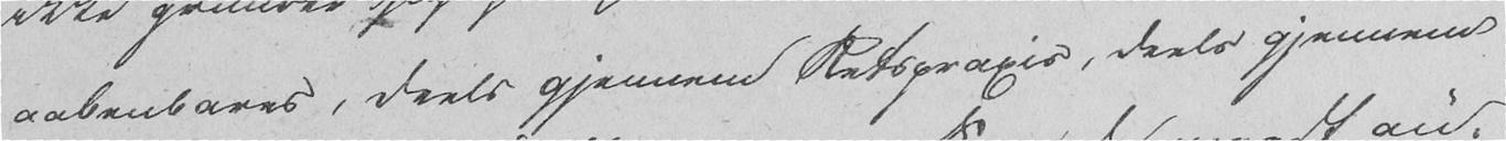aabenbares, deels gjennem Retspraxis, deels gjennem
